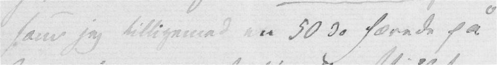som jeg tilligemed en 50 do hævede på
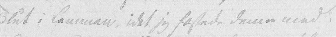lut i Lommen, idet jeg fæstede denne med
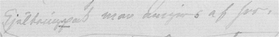Kjeltringpak man omgives af her,
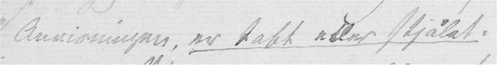Anvisningen, er tabt eller stjålet.
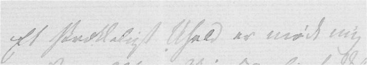Et skrækkeligt Uheld er mødt mig
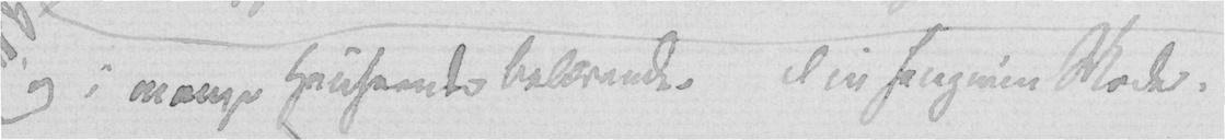og i mange Henseende belærende. Din hengivne Moder.
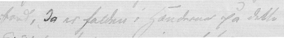bord, da er falden i Hænderne på dette
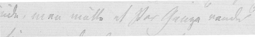ude, men måtte et Par Gange vende
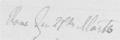Rom den 27de Marts
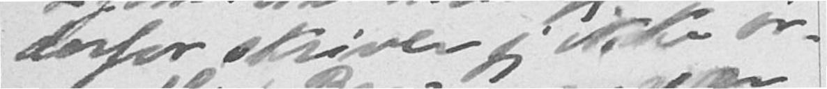derfor skriver jeg ikke or-
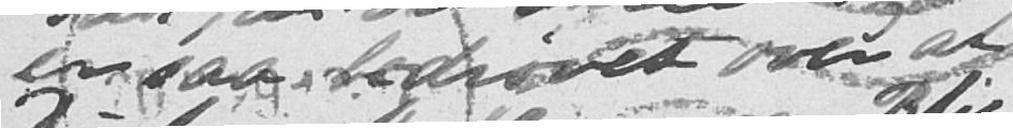er saa bedrøvet over at
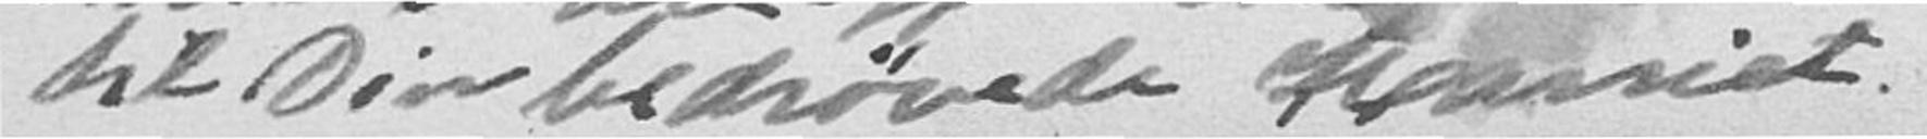til Din bedrøvede Harriet.
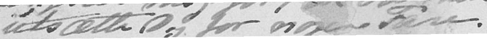utsætte Dig for nogen Fare.
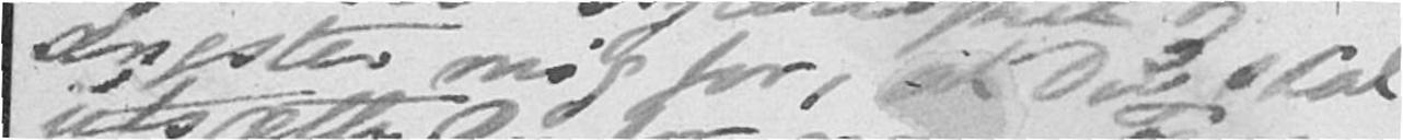ængster mig for, at Du stal
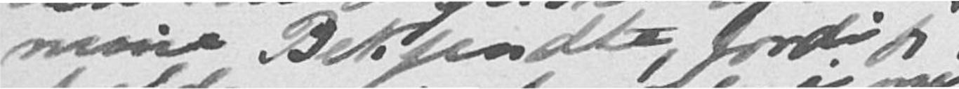mine Bekjendte, fordi jeg
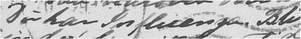Du har Influenza. Bli
